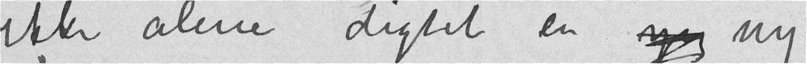ikkke alene digtet en  ny
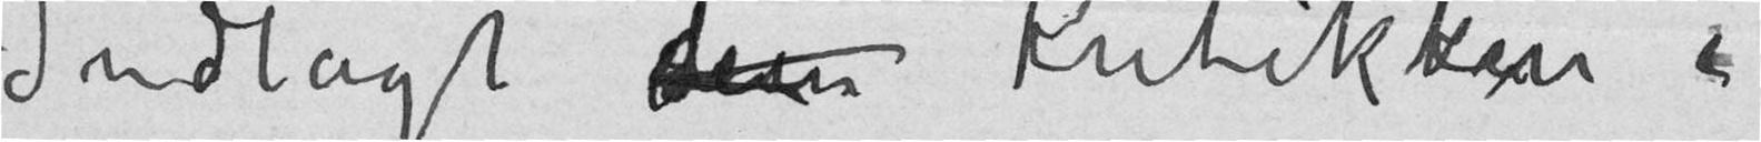Indlagt dem Kritikken i
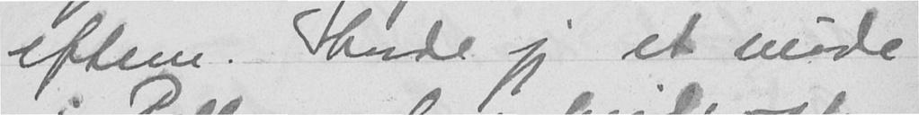efterm. havde jeg et møde
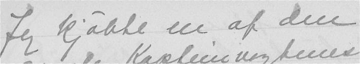Jeg kjøbte en af den
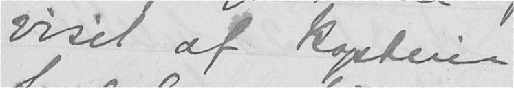visit af kaptein
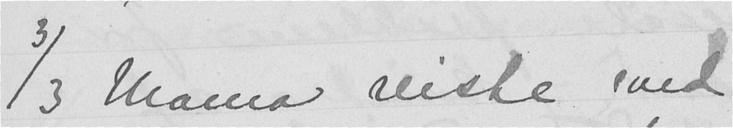3/3 Mama reiste med
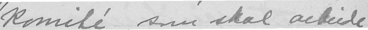komité, som skal arbeide
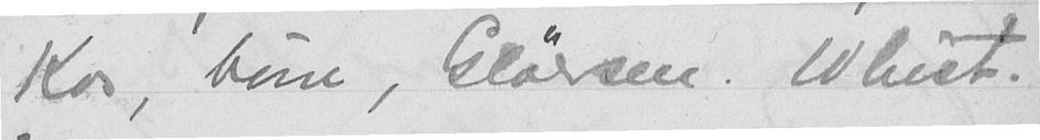Kos, børn, Gløersen. Whist.
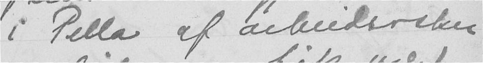i Pella af arbeidersker
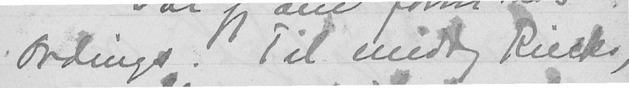Ordings. Til middag Riecks,
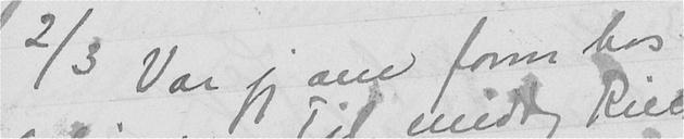2/3 Var jeg om form hos
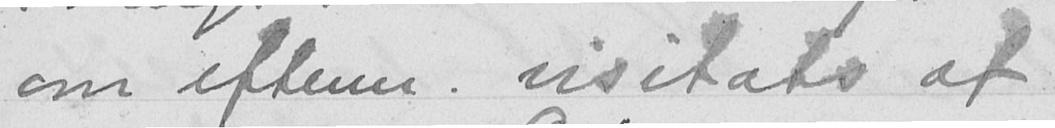om efterm. visitats af
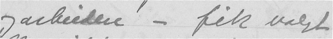og arbeidere - fik valgt
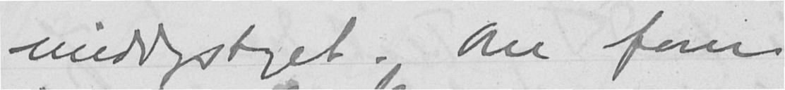middagstoget. Om form
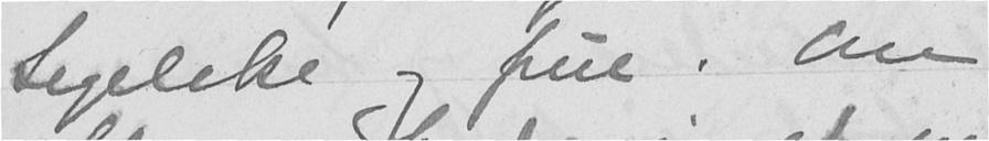Segelcke og frue. Om
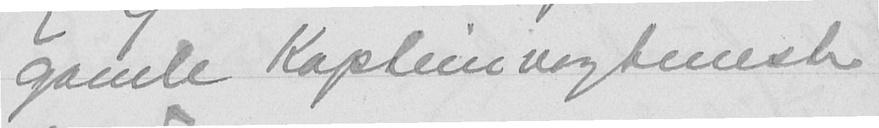gamle Kapteinvagtmester
